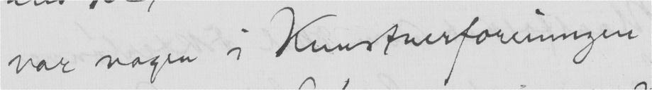var nogen i Kunstnerforeningen
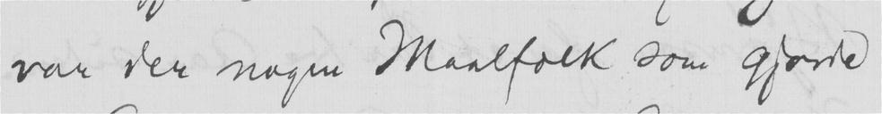var der nogen Maalfolk som gjorde
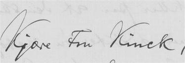Kjære Fru Kinck,
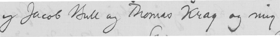og Jacob Bull og Thomas Krag og mig
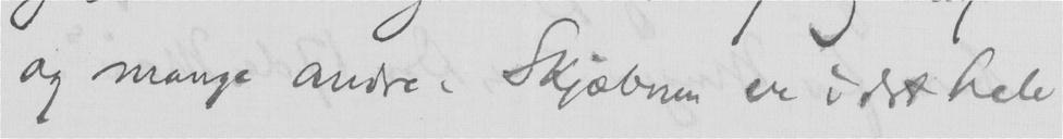og mange andre. Skjæbnen er i det hele
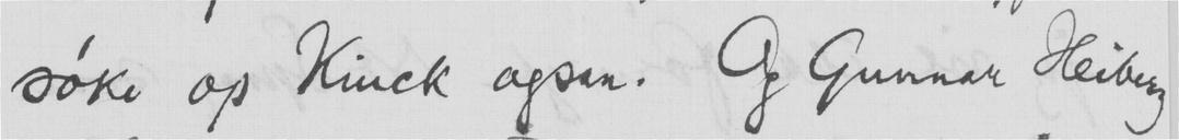søke op Kinck ogsaa. Og Gunnar Heiberg
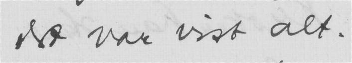det var vist alt.
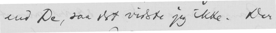end De, saa det vidste jeg ikke. Der
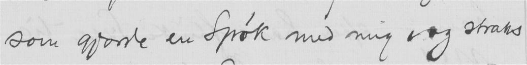som gjorde en Spøk med mig rog straks
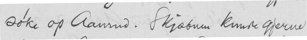søke op Aanrud. Skjæbnen kunde gjerne
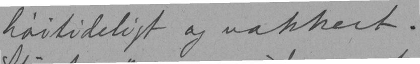høitideligt og vakkert.
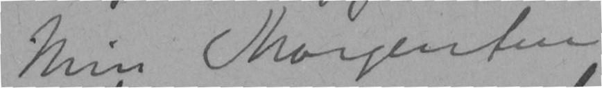Min Morgentur
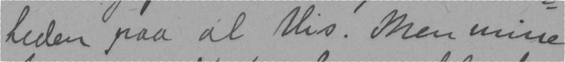heden paa al Vis. Men mine
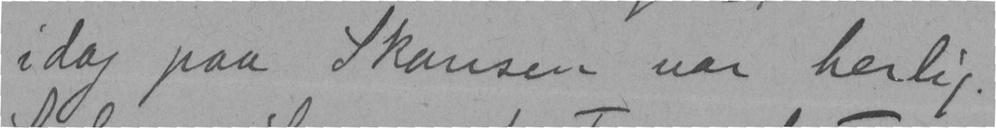idag paa Skansen var herlig.
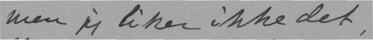men jeg liker ikke det.
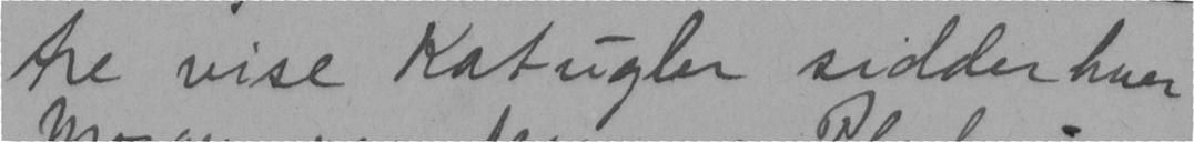tre vise Katugler sidder hver
